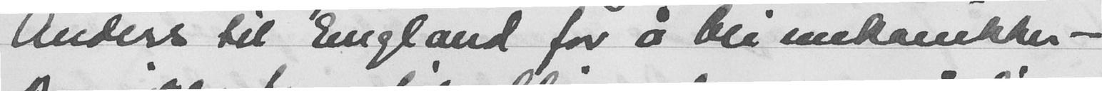Anders til England for å bli mekanikker -
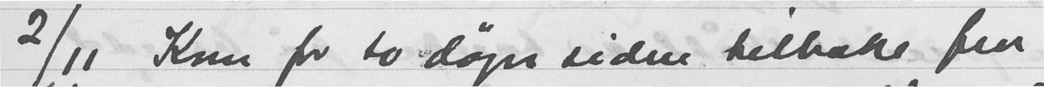2/11 Kom for to døgn siden tilbake fra
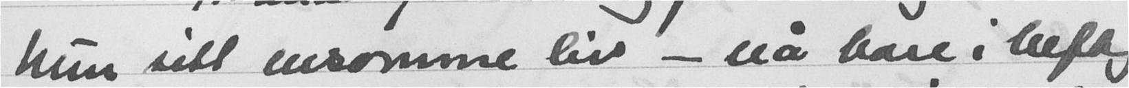hun sitt ensomme liv - nå bare i heftig
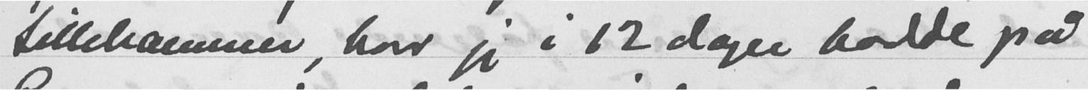Lillehammer, hvor jeg i 12 dager bodde på
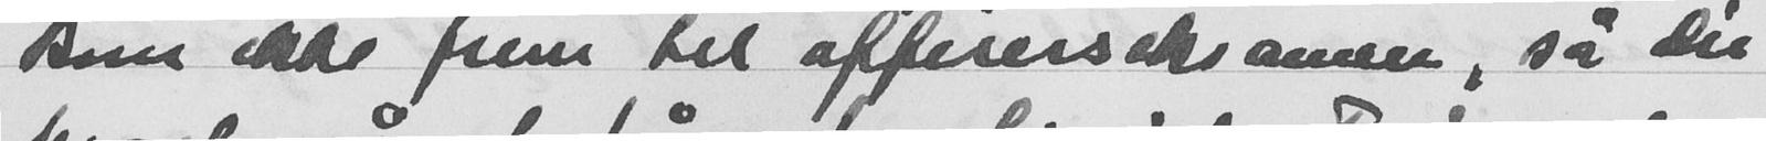kom ikke frem til offiserseksamen, så der
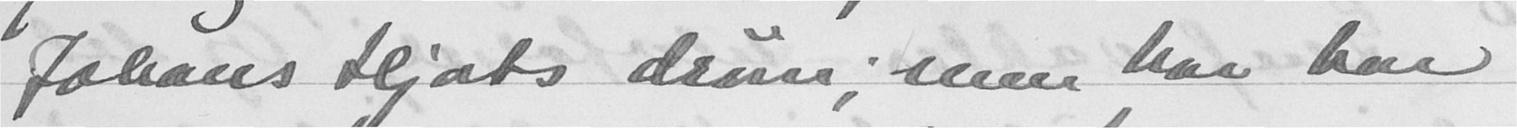Johans Hjorts drøm; men har har
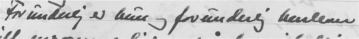Forunderlig er hun og forunderlig bursleaer
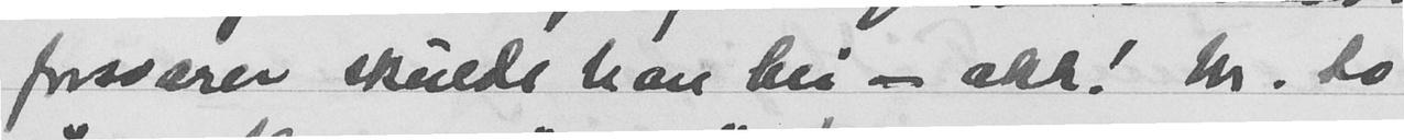forsvarer skulde han bli - akk! M. to
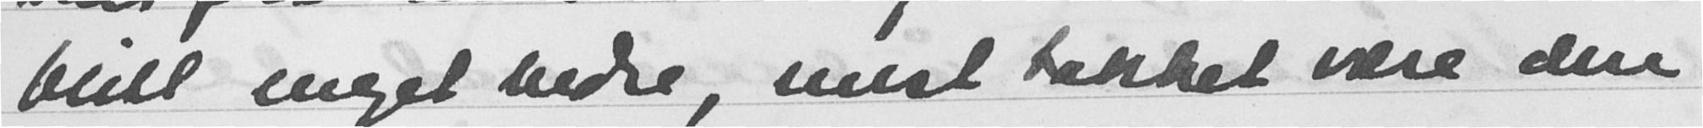blitt meget bedre, mest takket være den
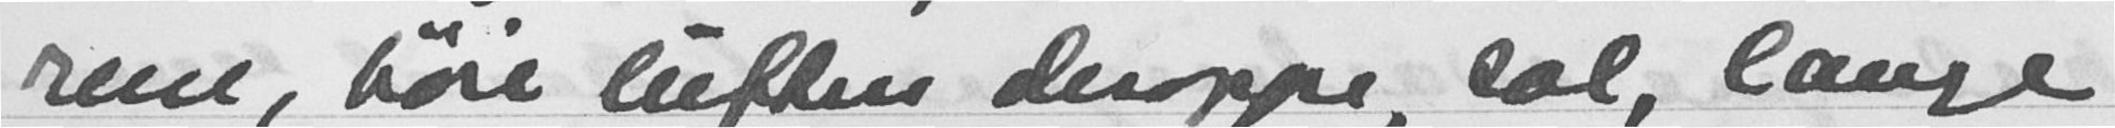rene, høie luften deroppe, sol, lange
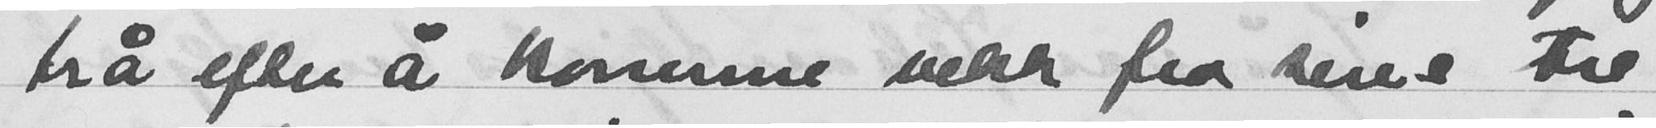trå efter å komme vekk fra sine tre
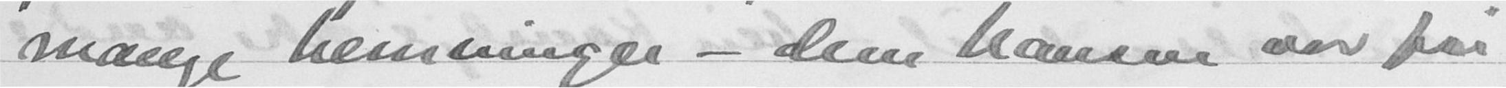mange hemninger - dem Nansen var fri
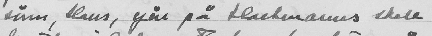sønn, Hans, går på Hartmanns skole
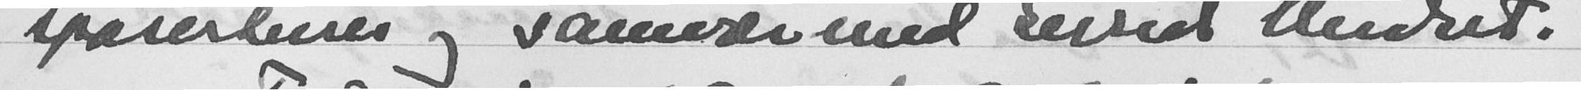spaserturer og samvær med Sigrid Undset.
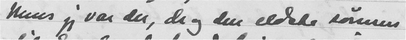Mens jeg var der, drog den eldste sønnen
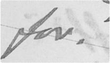for.
